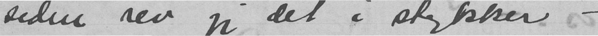siden rev jeg det i stykker -
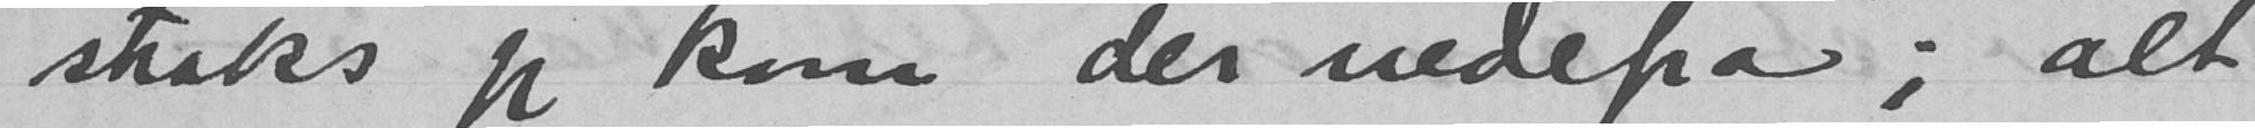straks jeg kom der nedefra; alt
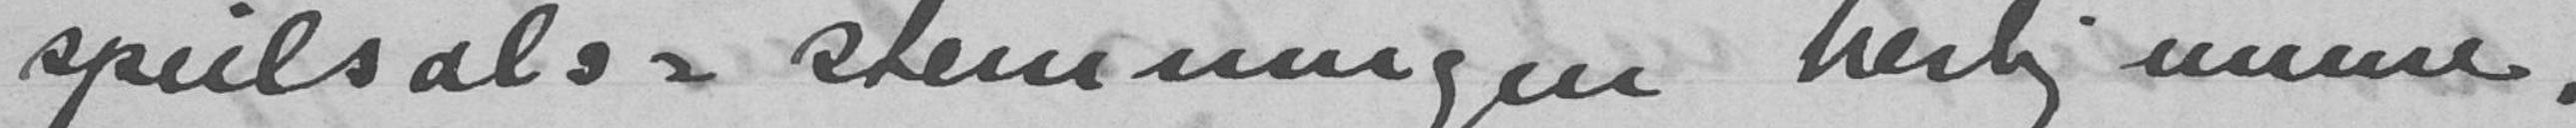speilsals-stemningen herhjemme,
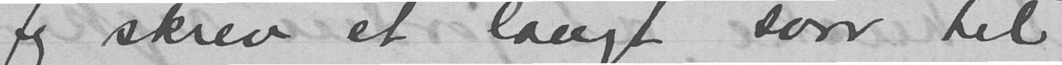Jeg skrev et langt svar til
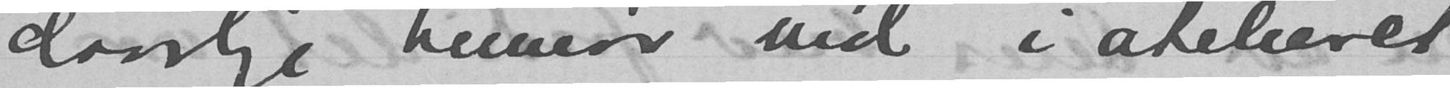daarlige humør ind i atelieret
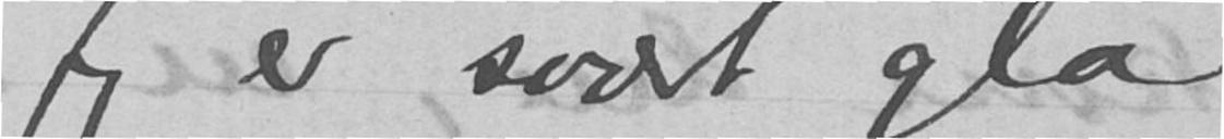Jeg er svært gla
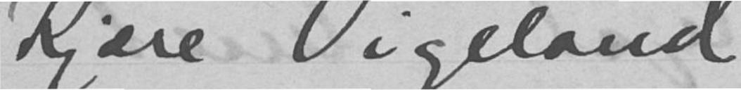Kjære Vigeland.
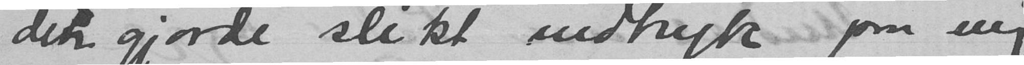den gjorde slikt indtryk paa mig
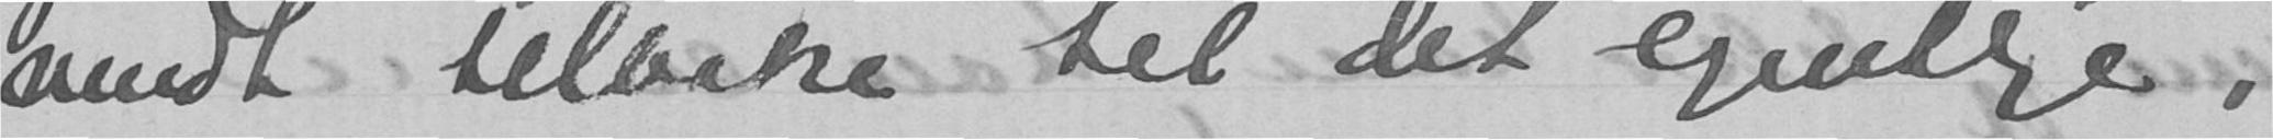vendt tilbake til det egentlige,
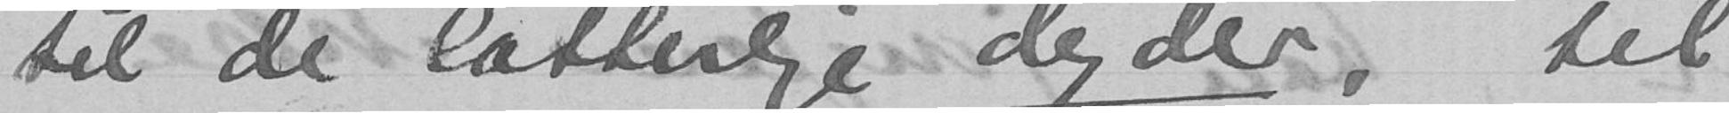til de latterlige dyder, til
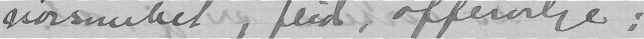nøisomhet, flid, offervilje;
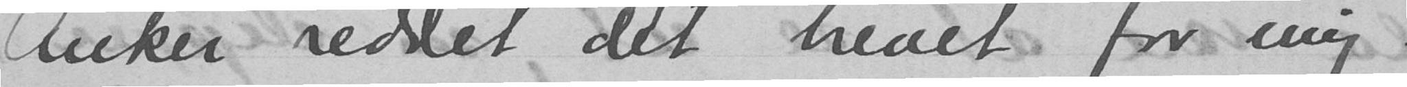Anker reddet det brevet for mig.
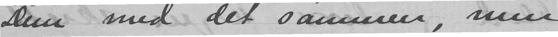Dem med det samme, men
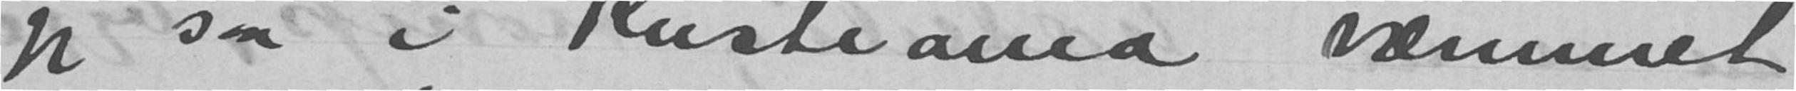jeg saa i Kristiania væmmet
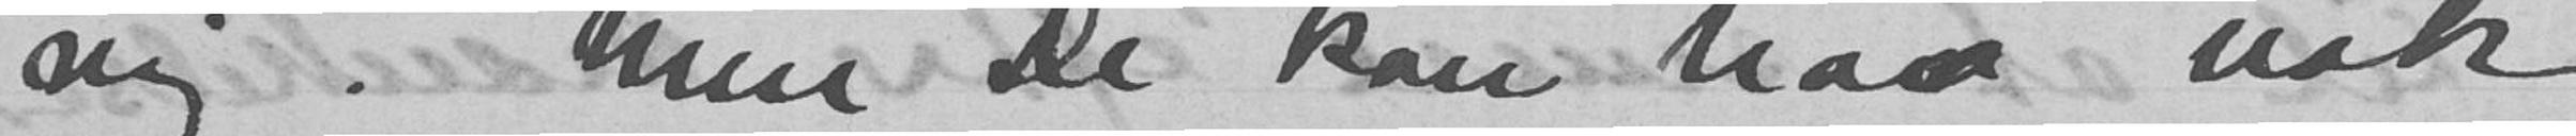mig. Men De kan ha nok
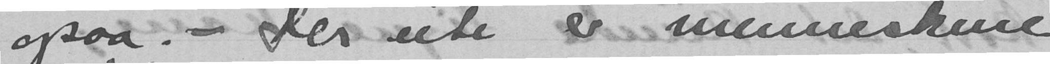ogsaa. - Der ute er menneskene
